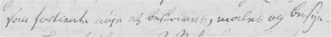som fortiente nøje at beskrives, males og besyn-
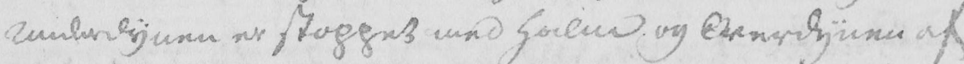Underdynen er stoppet med Halm og Overdynen af
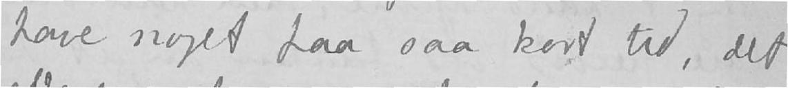have noget paa saa kort tid, det
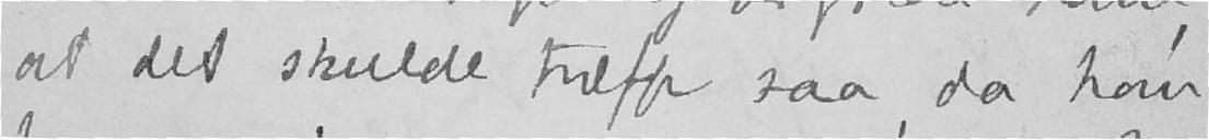at det skulde træffe saa, da han
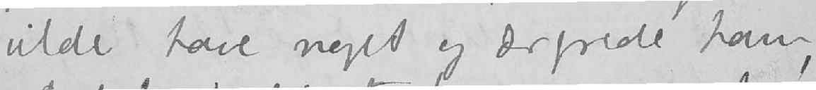vilde have noget og ærgrede ham
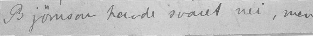Bjørnson havde svaret nei, men
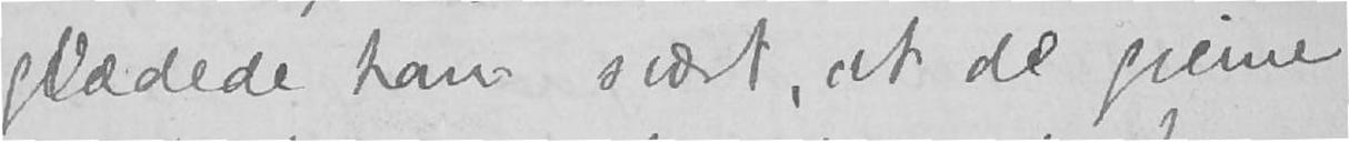glædede ham svært, at de gierne
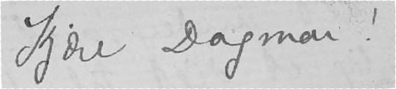Kjære Dagmar!
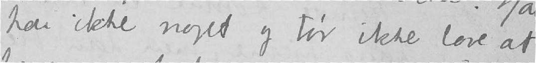har ikke noget og tør ikke love at
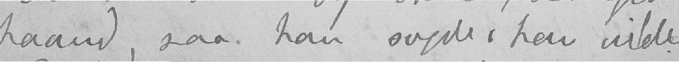haand, saa han sagde, han vilde
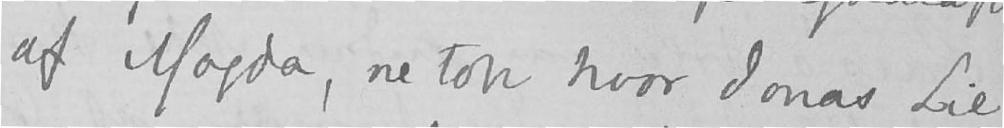af Magda, netop hvor Jonas Lie
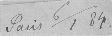Paris 6/1 84
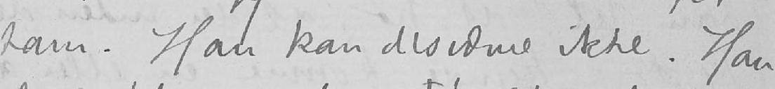ham. Han kan desværre ikke. Han
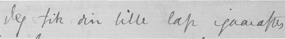Jeg fik din lille lap igaaraftes
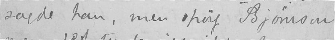sagde han, men spørg Bjørnson,
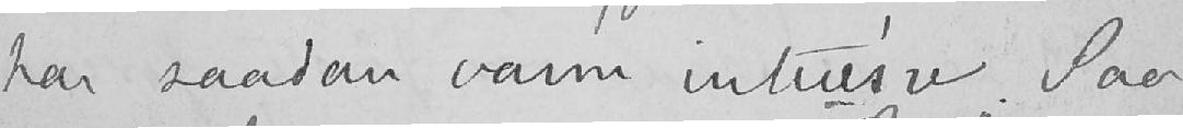har saadan varm interesse. Saa
